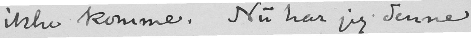ikke komme. Nu har jeg denne
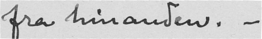fra hinanden. -
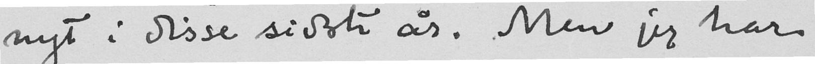nyt i disse sidste år. Men jeg har
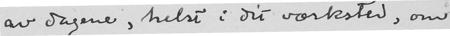av dagene, helst i dit værksted, om
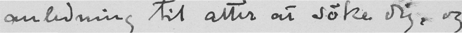anledning til atter at søke dig, og
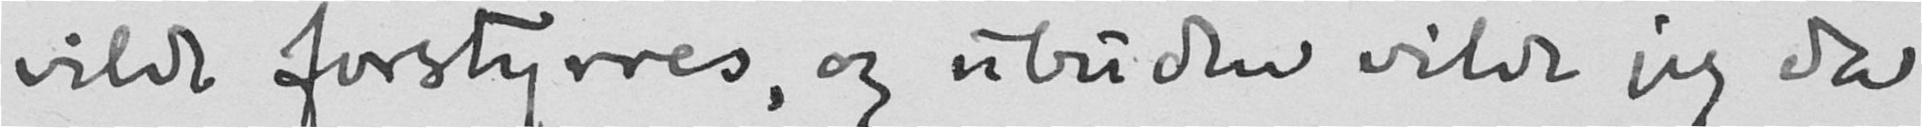vilde forstyrres, og ubuden vilde jeg da
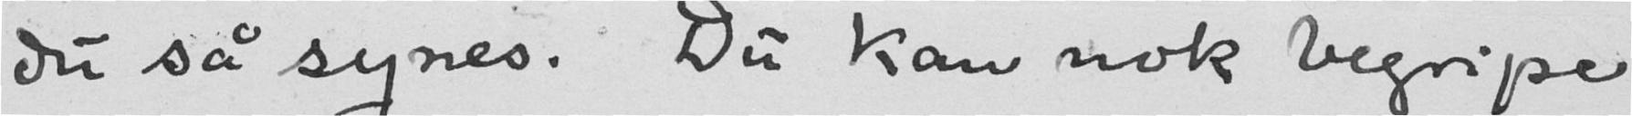du så synes. Du kan nok begripe
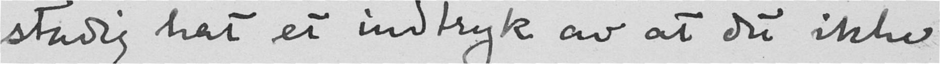stadig hat et indtryk av at du ikke
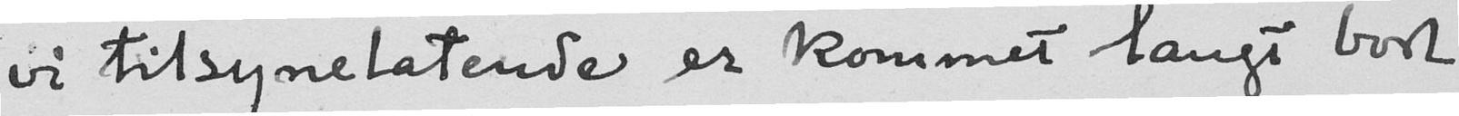vi tilsynelatende er kommet langt bort
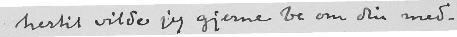hertil vilde jeg gjerne be om din med-
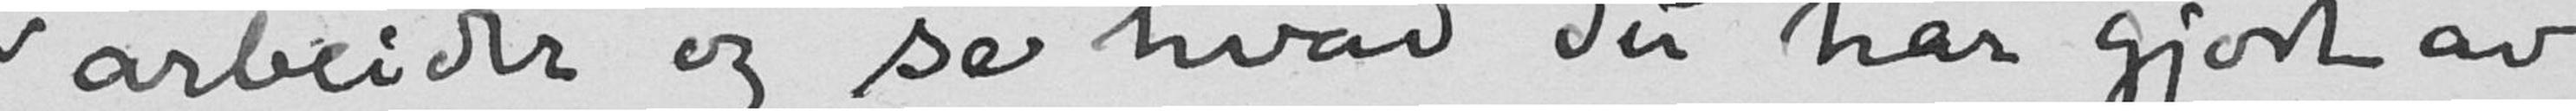arbeider og se hvad du har gjort av
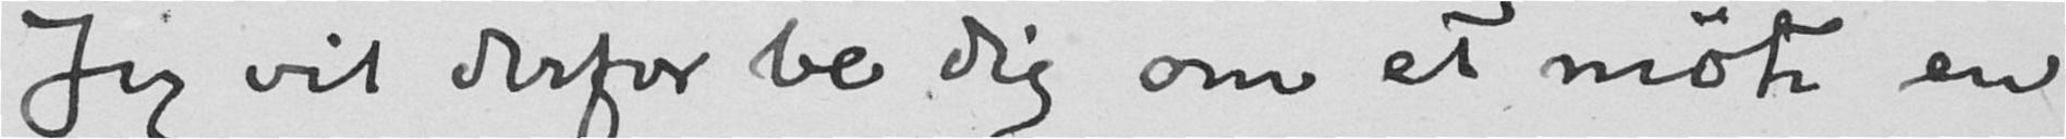Jeg vil derfor be dig om et møte en
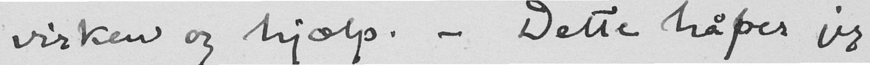virken og hjælp. - Dette håper jeg
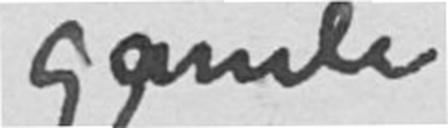gamle
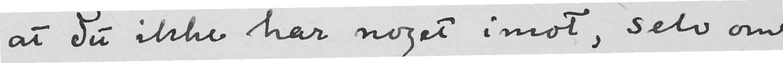at du ikke har noget imot, selv om
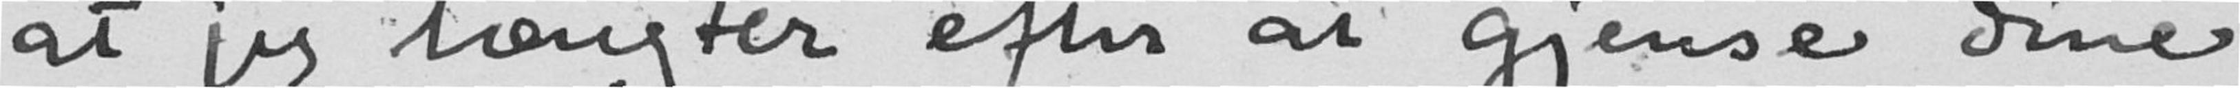at jeg længter efter at gjense dine
